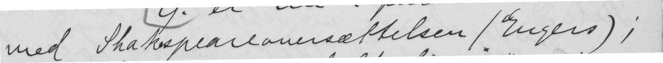med Shakspeareoversættelsen (Engers);
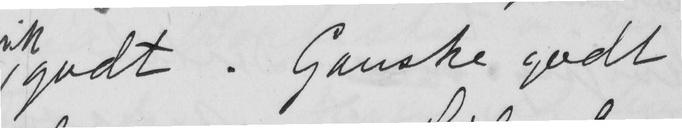godt. Ganske godt
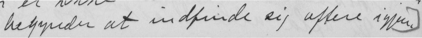begynder at indfinde sig oftere igjen.
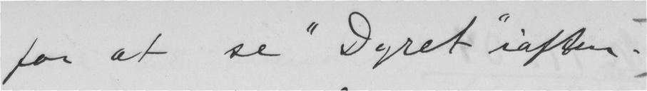for at se "Dyret" iaften.
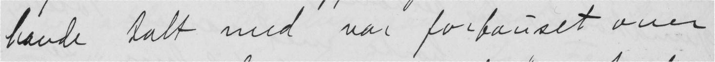havde talt med var forbauset over
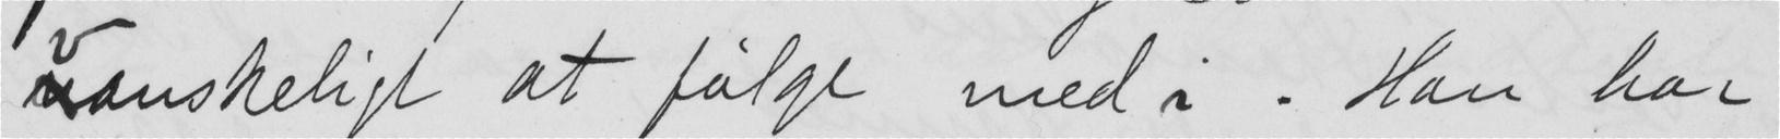vanskeligt at følge med i. Han har
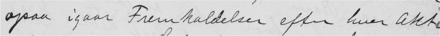ogsaa igaar Fremkaldelser efter hver Akt.
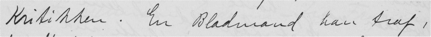Kritikken. En Bladmand han traf,
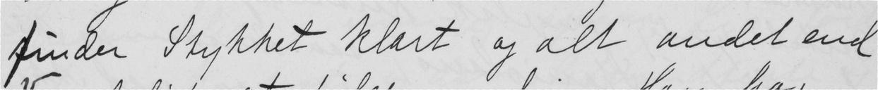finder Stykket klart og alt andet end
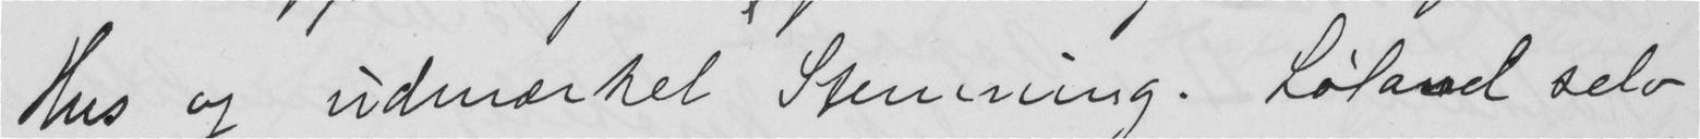Hus og udmærket Stemning. Løland selv
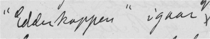"Edderkoppe"n igaar
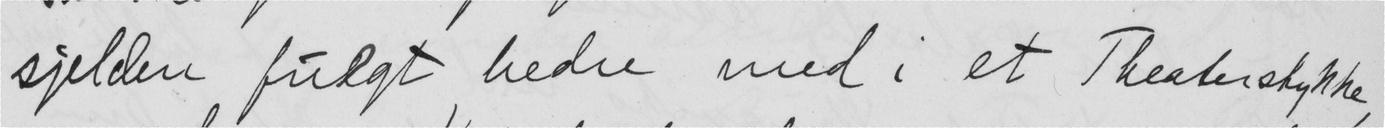sjelden fulgt bedre med i et Theaterstykke,
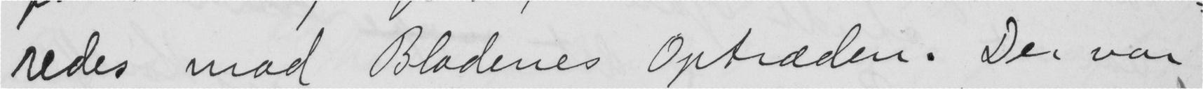redes mod Bladenes Optræden. Der var
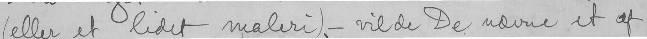(eller et lidet maleri), - vilde De nævne et af
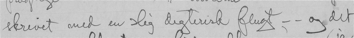skrivet med en slig digterisk flugt -- og det
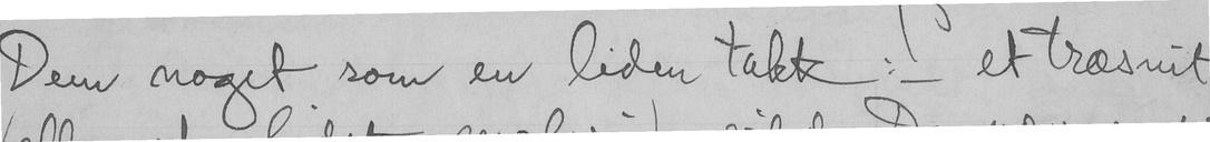Dem noget som en liden takk! - et træsnit
